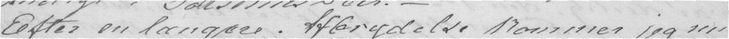Efter en længere Afbrytelse kommer jeg nu
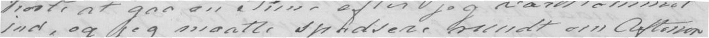ind, og jeg maatte spadsere rundt om Aftene
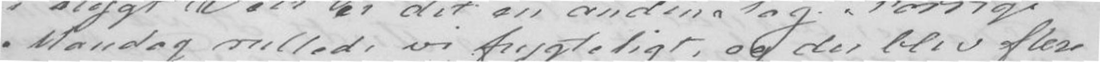Mandag rullede vi frygteligt, og der blev flere
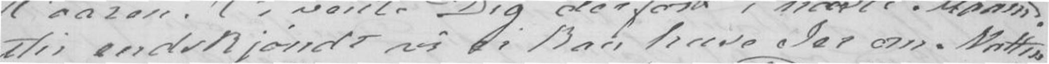thi endskjøndt vi ei kan have Jer om Natten,
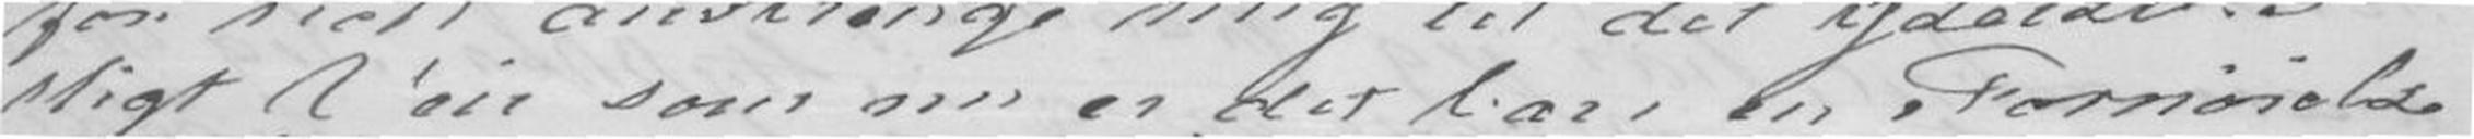sligt Veir som nu er det bare en Fornøielse
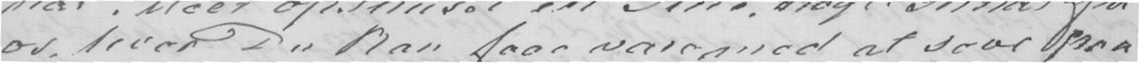os, hvor Du kan faae være med at sove paa
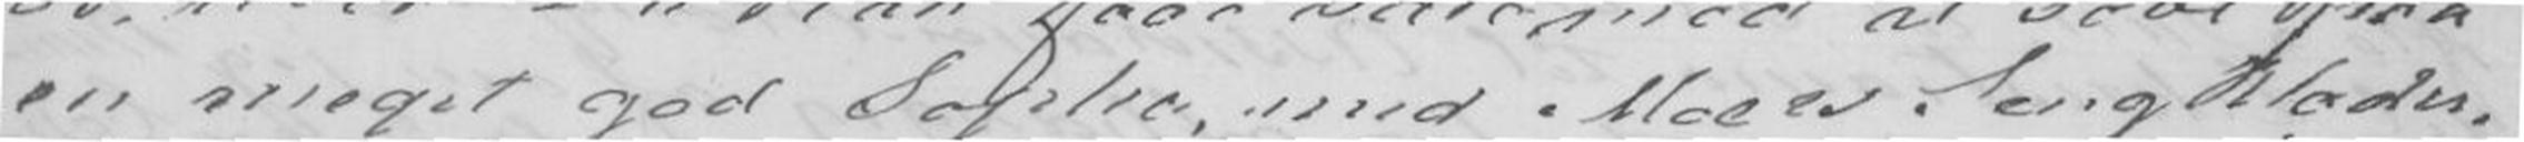en meget god Sopha, med Moers Sengklæder,
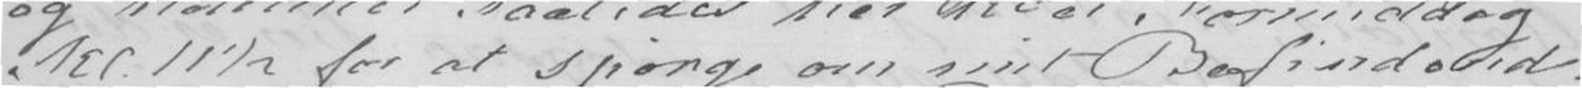Kl.11½ for at spørge om mit Bifindend
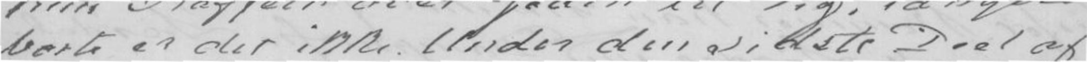borte er det ikke. Under den sidste Deel af
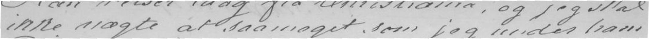ikke nægte at saameget som jeg under ham
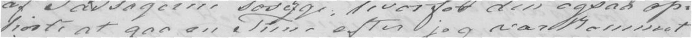hørte at gaa en Time efter jeg var kommet
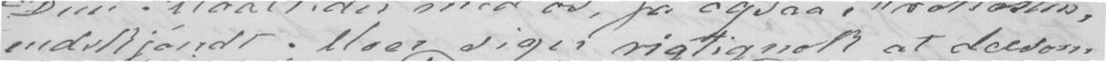endskjøndt Moer siger rigtignok at dersom
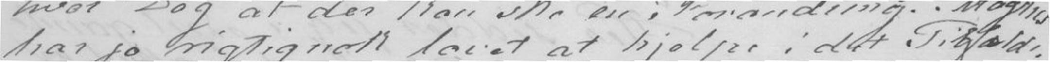har jo rigtignok lovet at hjelpe i det Tilfælde,
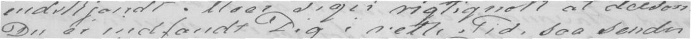Du ei indfandt Dig i rette Tid, saa sender
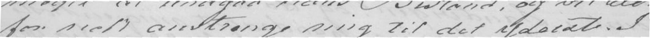for nok anstrenge mig til det yderste. I
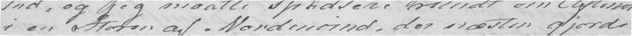i en Storm af Nordenvind, der næsten gjorde
